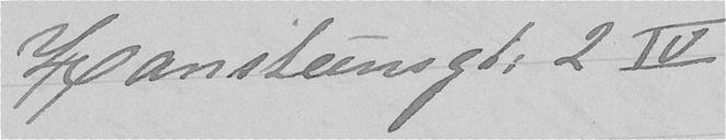Hansteensgt. 2 IV
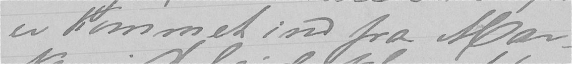er kommet ind fra Mar-
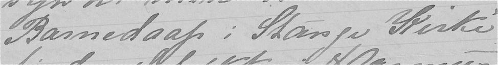Barnedaap i Stange Kirke
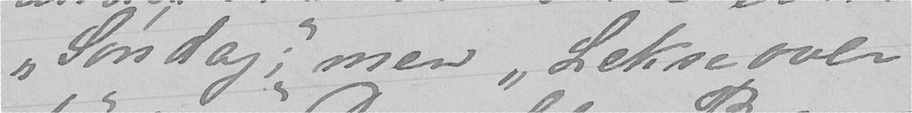Søndag; men Lekseover-
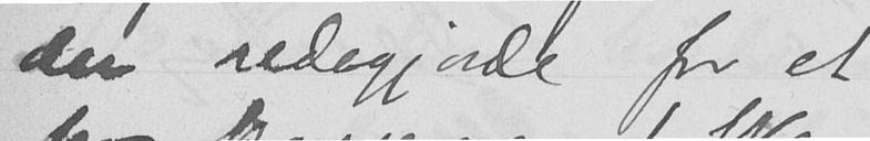der redegjorde for et
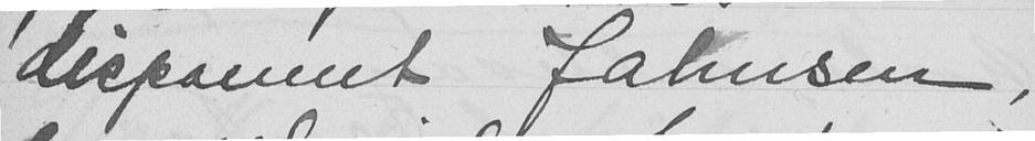disponent Johansen,
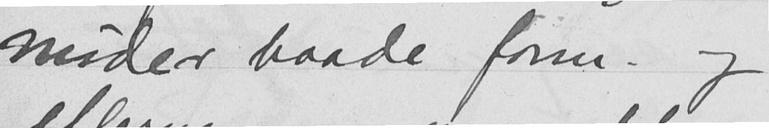møder baade form. og
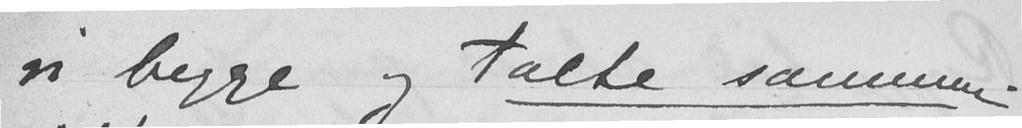vi begge og talte sammen.
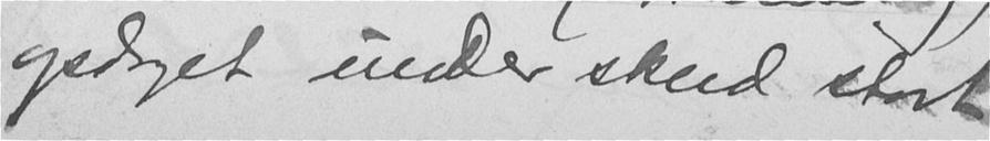opdaget underskud stort
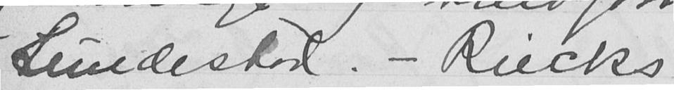Lundestad. - Riecks
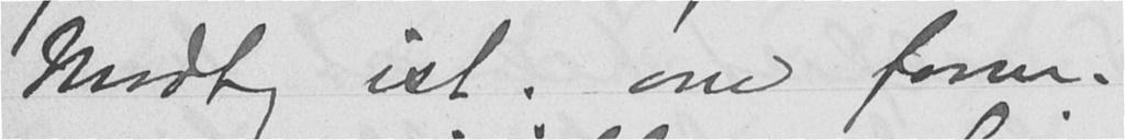Modtog ist. om form.
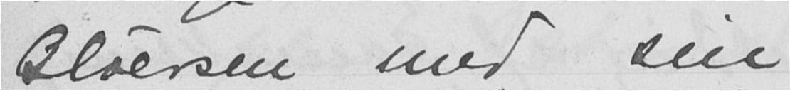Gløersen med sin
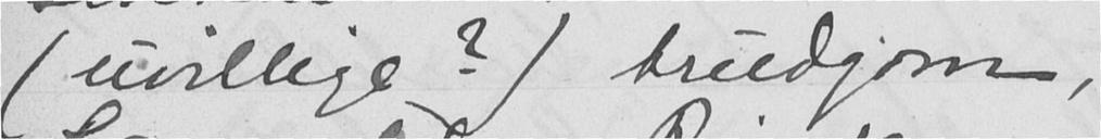(uvillige?) brudgom,
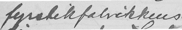fyrstikfabrikkens
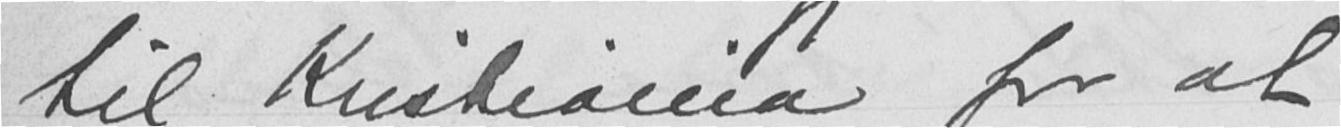til Kristiania for at
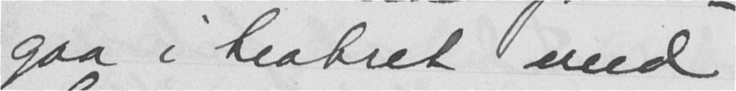gaa i teatret med
